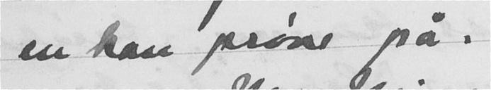en kan prøve på.
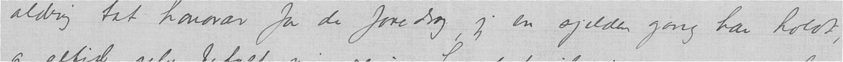aldrig tat honorar for de foredrag, jeg en sjelden gang har holdt,
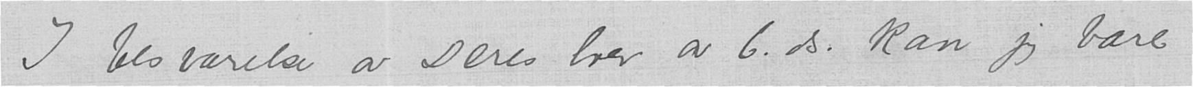I besvarelse av Deres brev av 6. ds. kan jeg bare
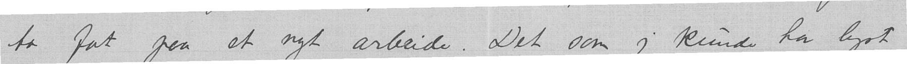ta fat paa et nyt arbeide. Det som jeg kunde ha lyst
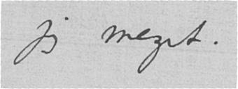jeg meget.
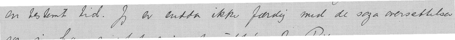en bestemt tid. Jeg er endda ikke færdig med de saga oversettelser
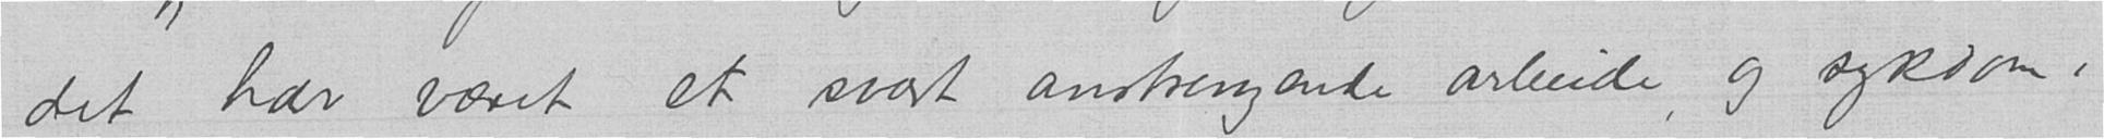det har været et svært anstrengende arbeide, og sykdom i
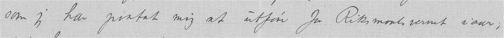som jeg har paatat mig at utføre for Riksmaalsvernet iaar;
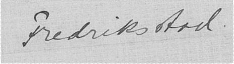Fredriksstad
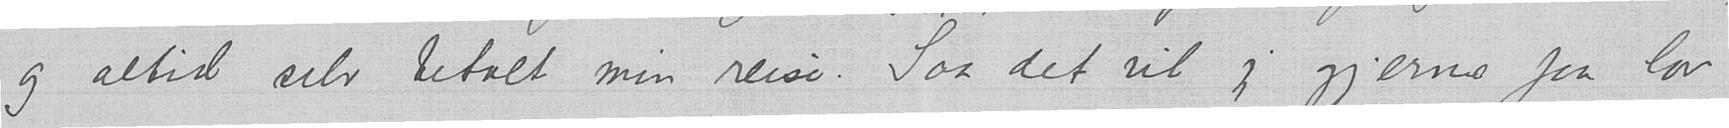og altid selv betalt min reise. Saa det vil jeg gjerne faa lov
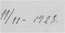11/11-1923
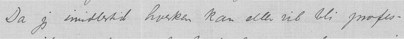Da jeg imidlertid hverken kan eller vil bli profes-
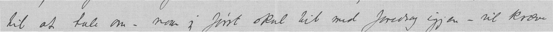til at tale om - naar jeg først skal til med foredrag igjen – vil kræve
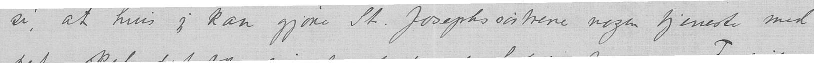si, at hvis jeg kan gjøre St. Josephssøstrene nogen tjeneste med
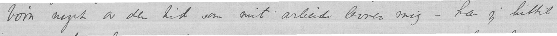børn noget av den tid som mit arbeide levner mig – har jeg hittil
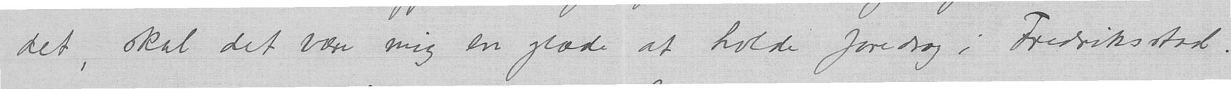det, skal det være mig en glæde at holde foredrag i Fredriksstad.
